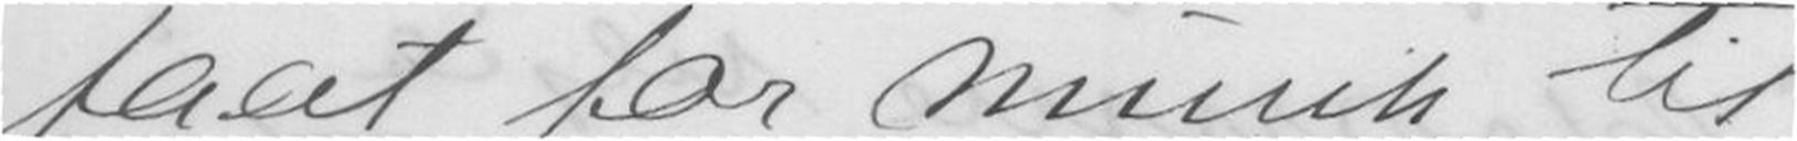faat for musik til
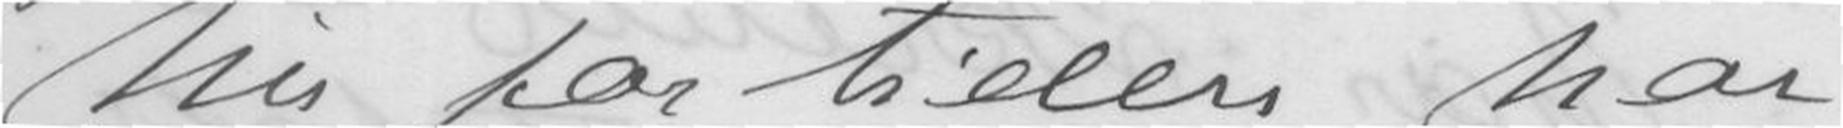Nu for tiden har
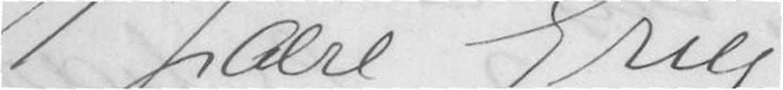Kjære Grieg
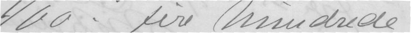400 fire hundrede -
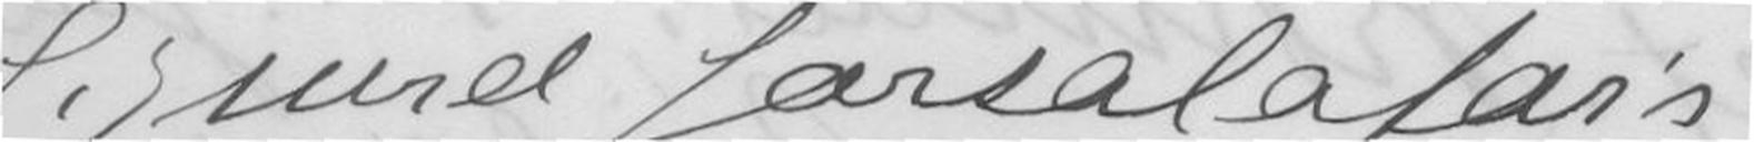Sigurd Jorsalfar's
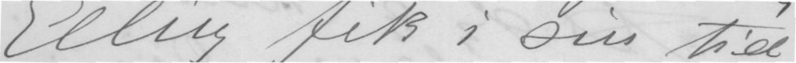Elling fik i sin tid
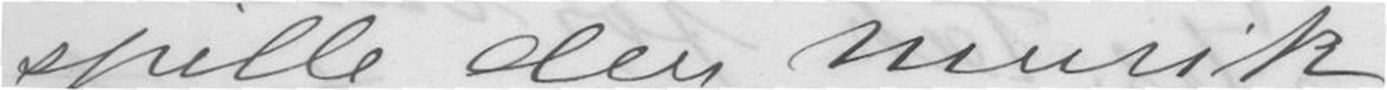spille den musik.
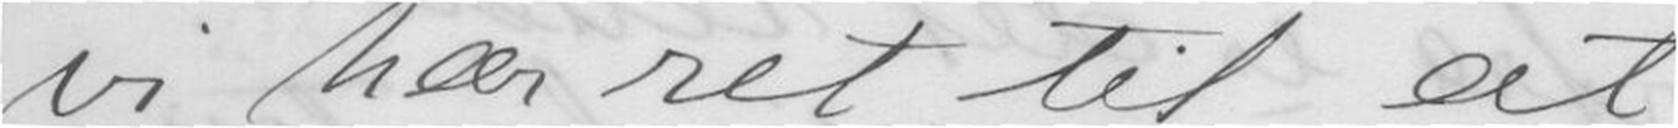vi har ret til at
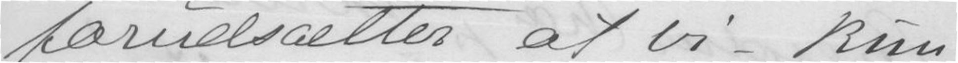forudsætter at vi - kun
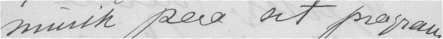musik paa sit program
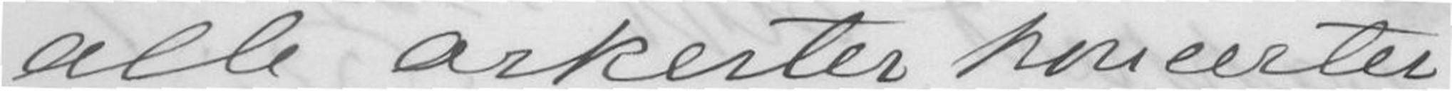alle orkester koncerter
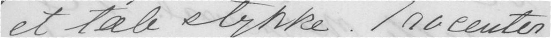et tale stykke. Trocenter
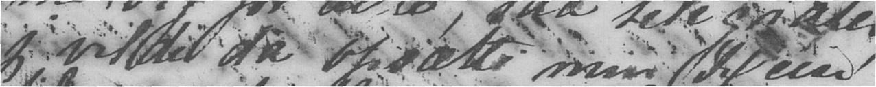jeg vilde da opsætte min Reise
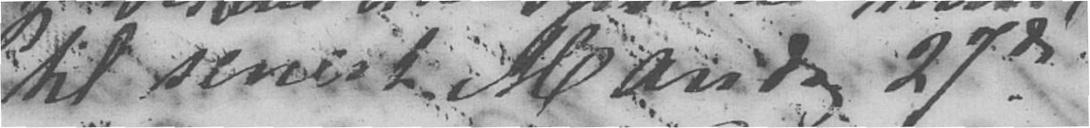til senest Mandag 27de.
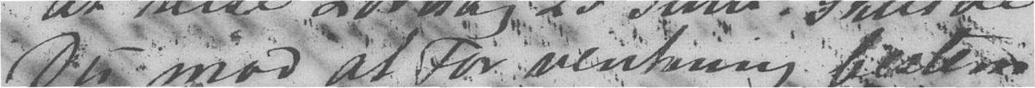Du mod al Forventning bestem-
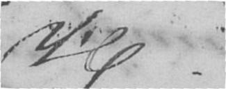H.
